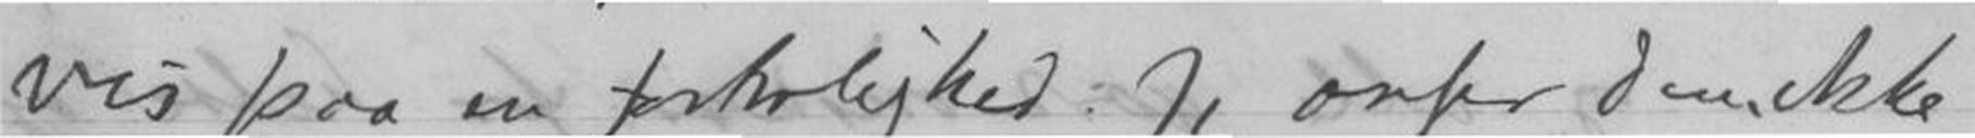vis paa en fortrolighed. Jeg anser dem ikke
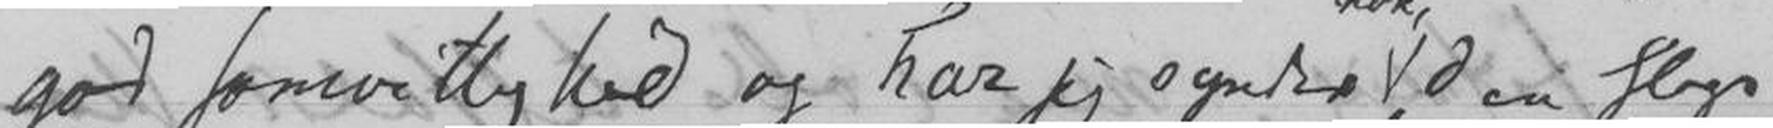god samvittighed og har jeg syndet den slags
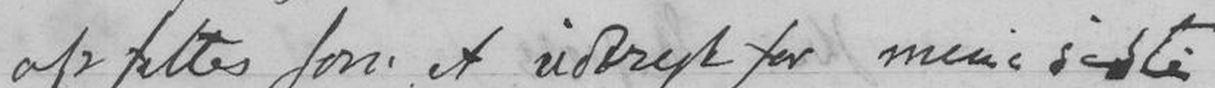opfattes som et udtryk for mine sidste
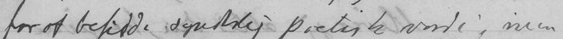for at besidde synderlig pratisk verdi, men
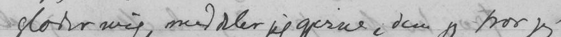glæder mig, medeler jeg gjerne, dem jeg tror jeg
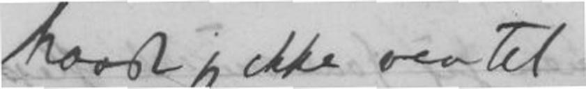havde ikke ventet.
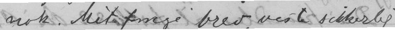nok. Mit forige brev, viste sikkerlig
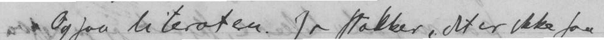Og saa literaten jo stakker, det er ikke saa
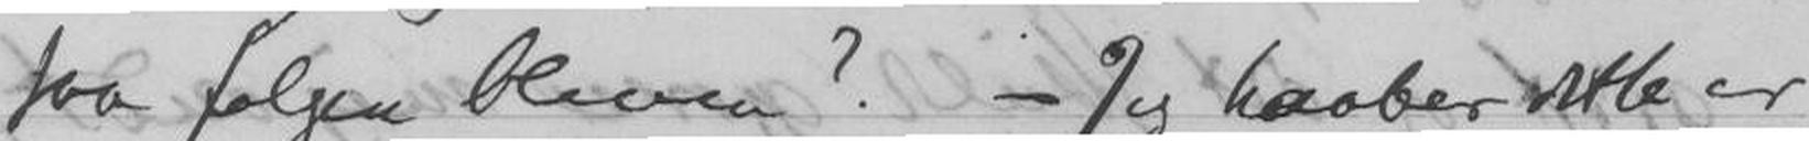saa følgen bleven? Jeg haaber dette er
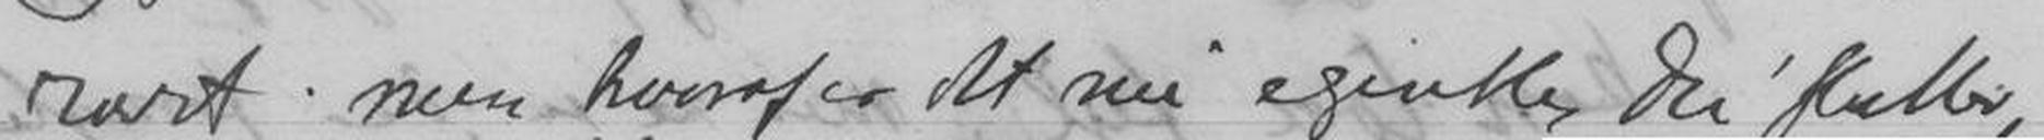rart, men hvoraf er det nu egentlig Du skulle,
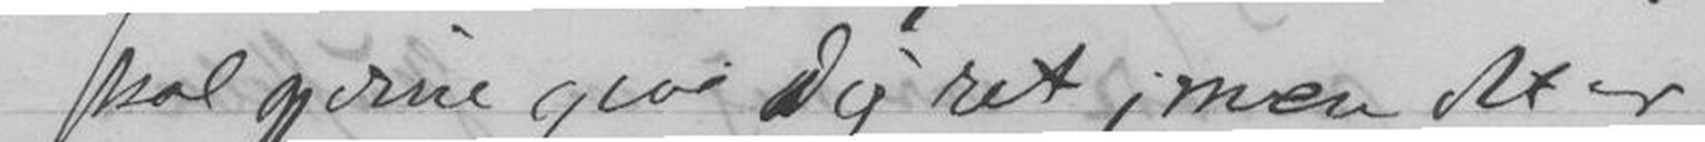skal gjerne give dig ret, men det er
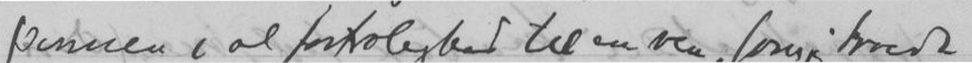pennen, al fortrolighed til en ven, som jeg troede
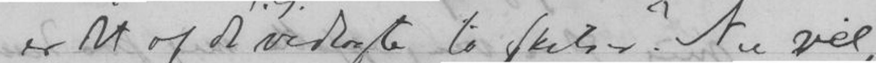er det af de vedlagte to skitser? Nu vel,
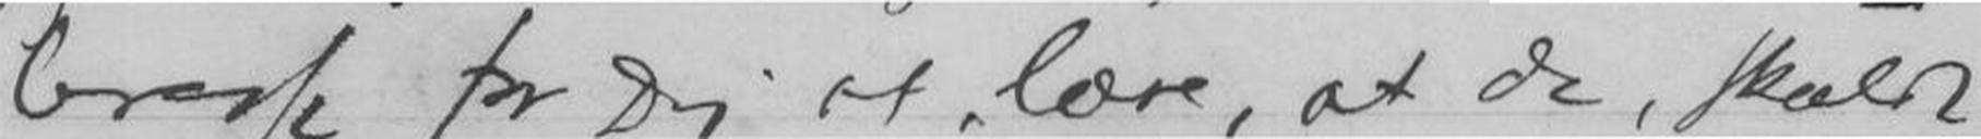tresse for Dig at "lære", at de, skulde
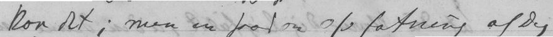kan det; men en saadan opfatning af Dig
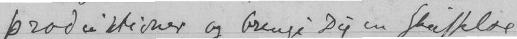productioner og bringe Dig en skufplas
